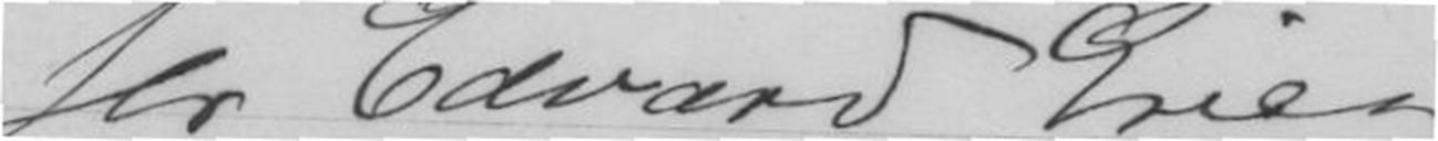Hr Edvard Grieg,
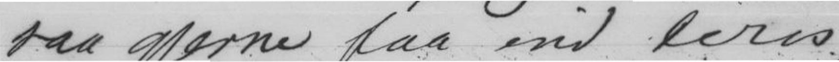saa gjerne faa ind Deres
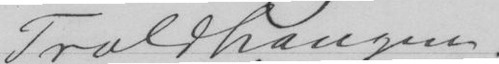Troldhaugen.
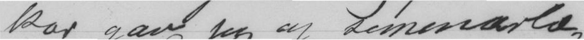kor gav jeg og semenarlæ-
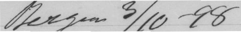Bergen 3/10 -98
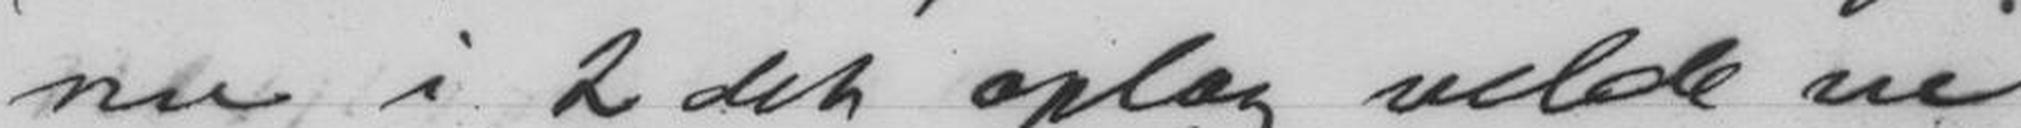nu i 2 det oplag vilde vi
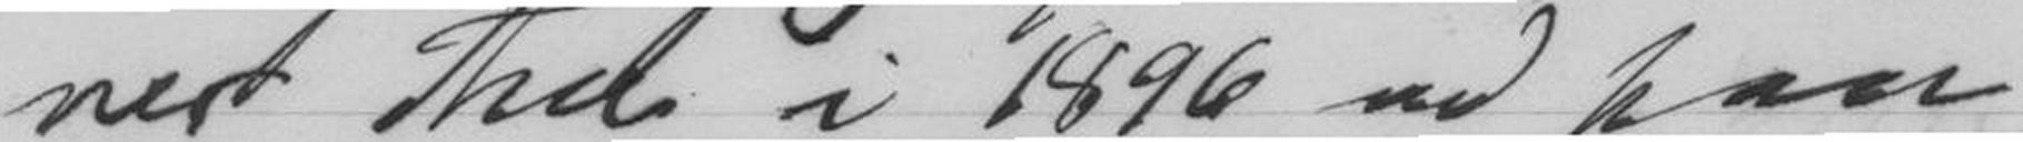rer Thu i 1896 ud paa
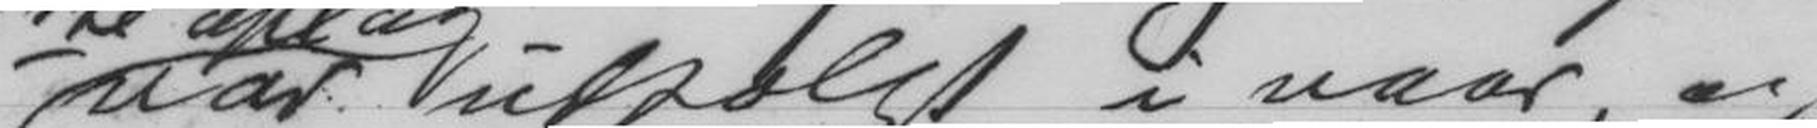1ste oplag var udsolgt i vaar, og
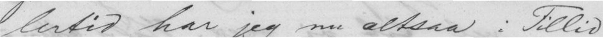lertid har jeg nu altsaa i Tillid
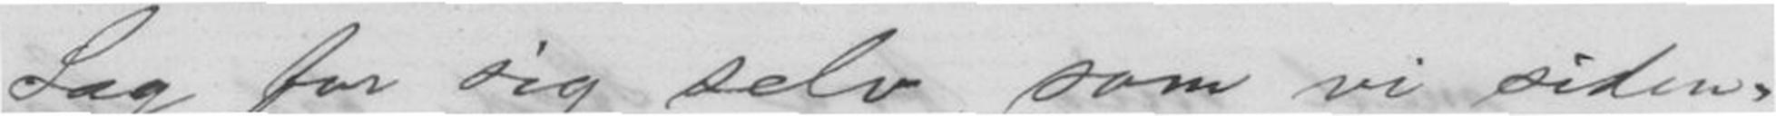Sag for sig selv, som vi siden-
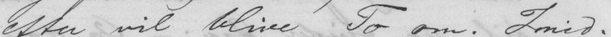efter vil blive To om. Imid-
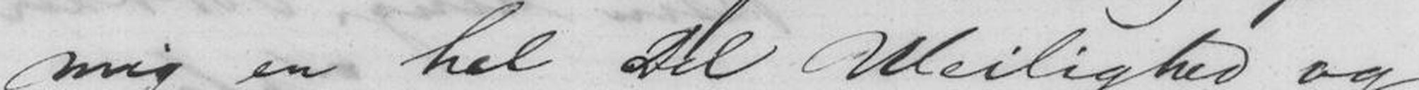mig en hel Del Uleilighed og
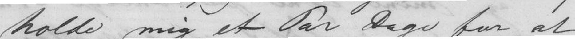holde mig et Par Dage for at
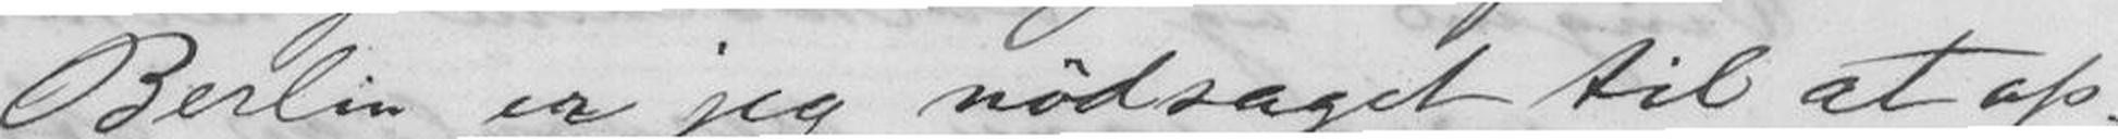Berlin er jeg nødsaget til at op-
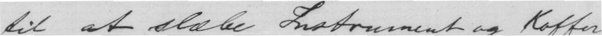til at slæbe Instrument og Koffer
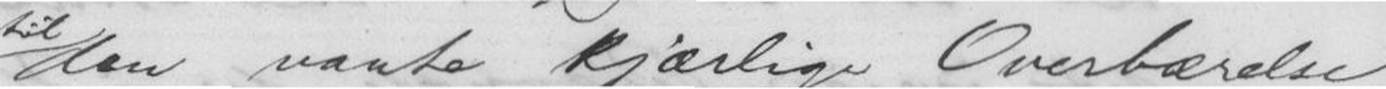til den vante kjærlige Overbærelse
